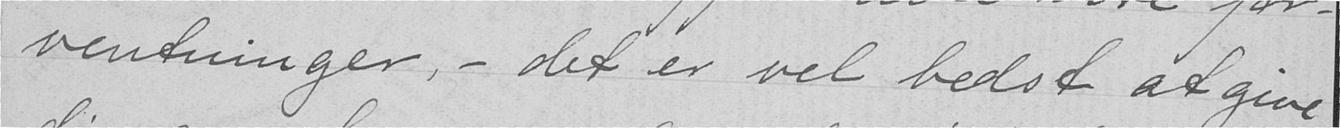ventninger, - det er vel bedst at give
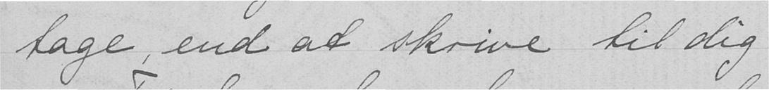tage end at skrive til dig.
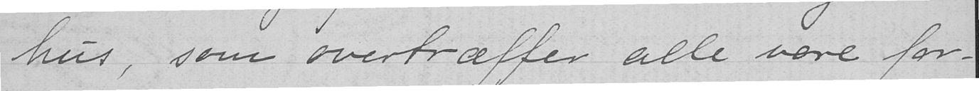hus, som overtræffer alle vore for-
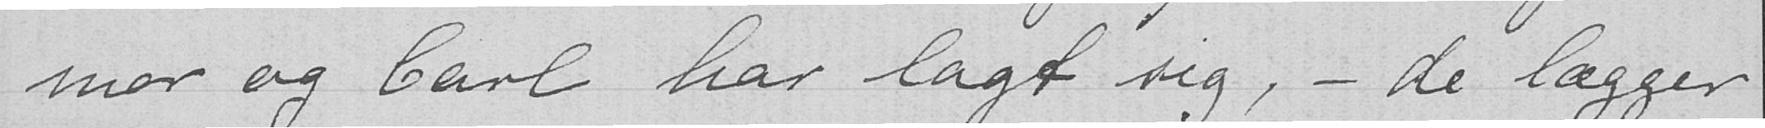mor og Carl har lagt sig, - de lægger
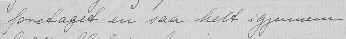foretaget en saa helt igjennem
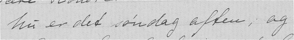Nu er det søndag aften; og
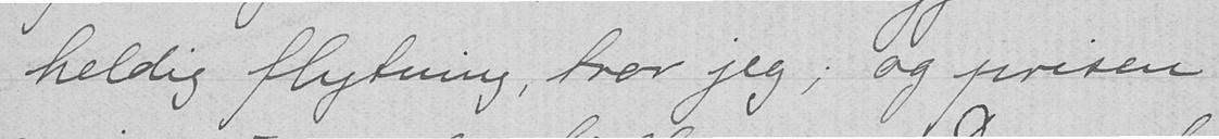heldig flytning, tror jeg; og prisen
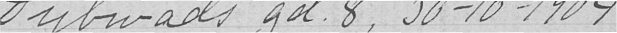Dybvads gd. 8, 30-10-1904
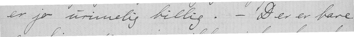er jo urimelig billig. - Der er bare
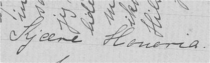Kjære Honoria!
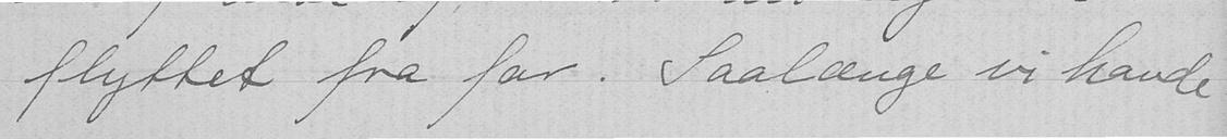flyttet fra far. Saalænge vi havde
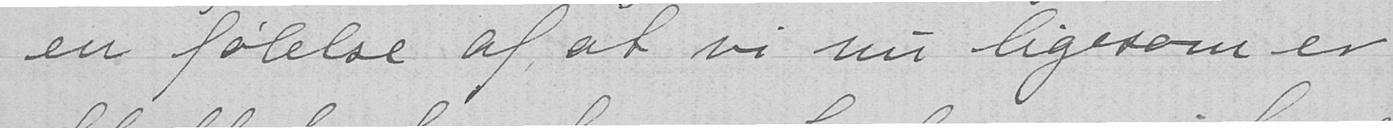en følelse af at vi nu ligesom er
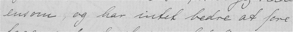ensom, og har intet bedre at fore-
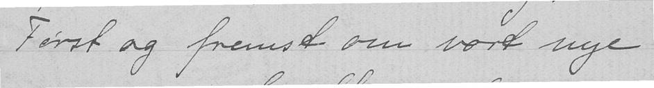Først og fremst om vort nye
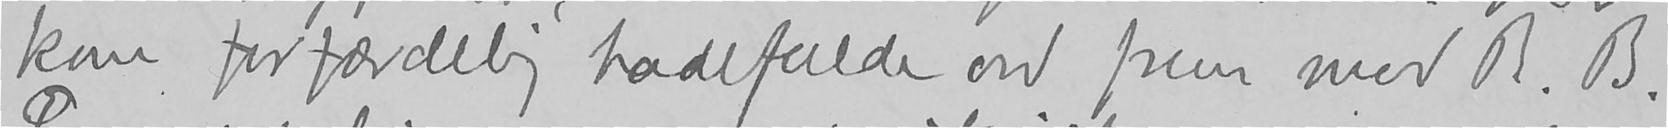kom forfærdelig hadefulde ord frem mod B. B.
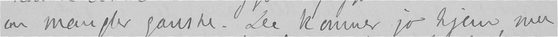en mangler ganske. De kommer jo hjem, men
